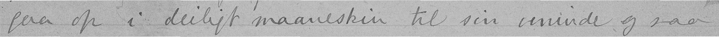gaa op i deiligt maaneskin til sin veninde og saa
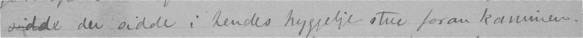sidde der sidde i hendes hyggelige stue foran kaminen.
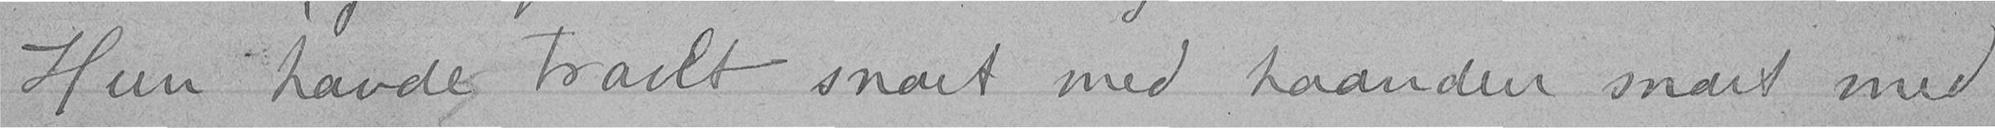Hun havde travlt snart med haanden snart med
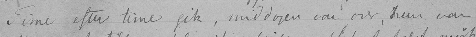Tirne efter time gik, middagen var over, hun var
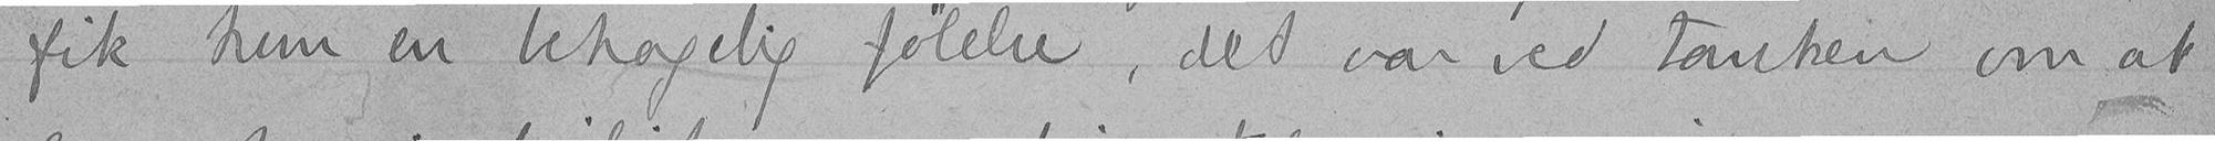fik hun en behagelig følelse, det var ved tanken om at
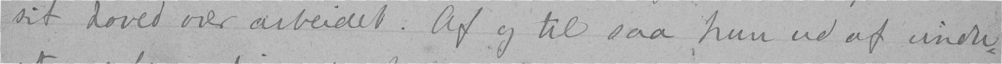sit hoved over arbeidet. Af og til saa hun ud af vindu-
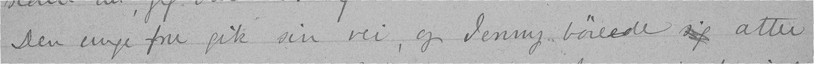Den unge fru gik sin vei, og Jenny bøieede sig atter
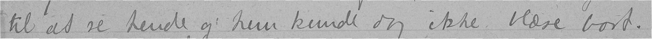til at se hende, og hun kunde dog ikke blæse bort.
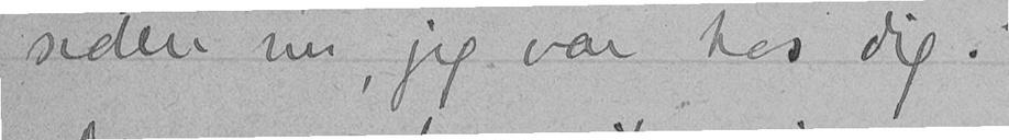siden nu, jeg var hos dig."
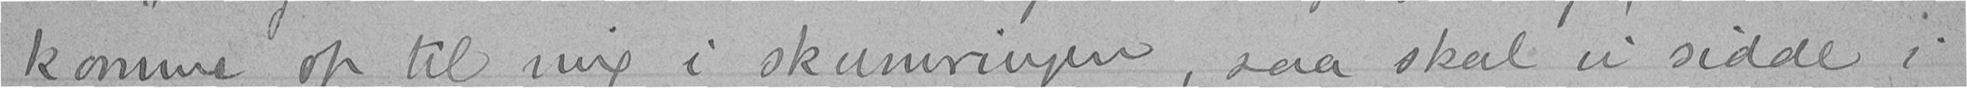komme op til mig i skumringen, saa skal vi sidde i
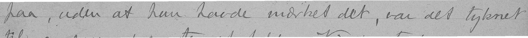paa, uden at hun havde mærket det, var det tyknet
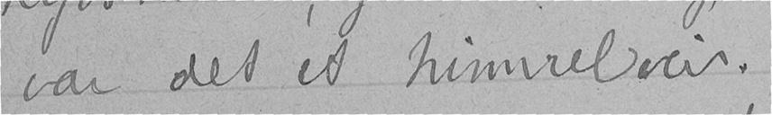var det et himmelveir.
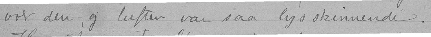over den, og luften var saa lys skinnende.
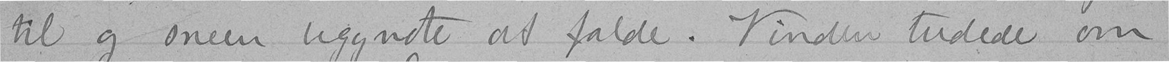til og sneen begyndte at falde. Vinden tudede om
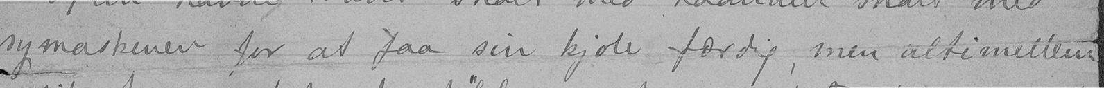symaskinen for at faa sin kjole færdig, men altimellem
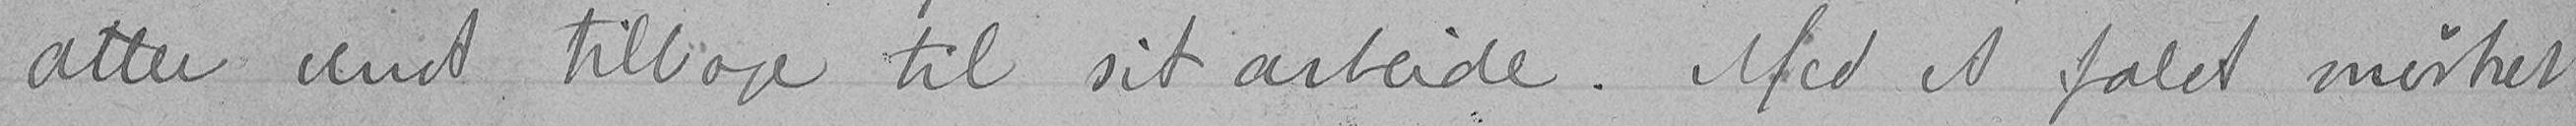atter vendt tilbage til sit arbeide. Med et faldt mørket
